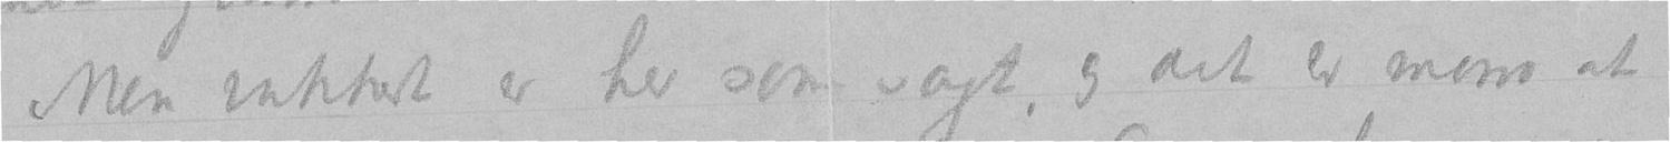Men vakkert er her som sagt, og det er morro at
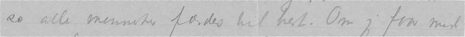se alle mennesker færdes til hest. Om jeg faar med
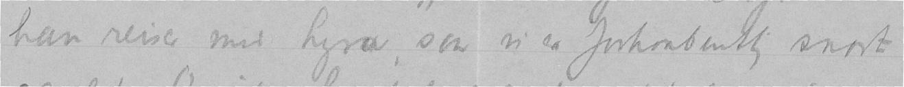han reiser med Lyra, saa vi er forhaabentlig snart
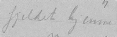fjeldet hjemme.
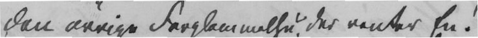den øvrige forglemmelse der venter En!
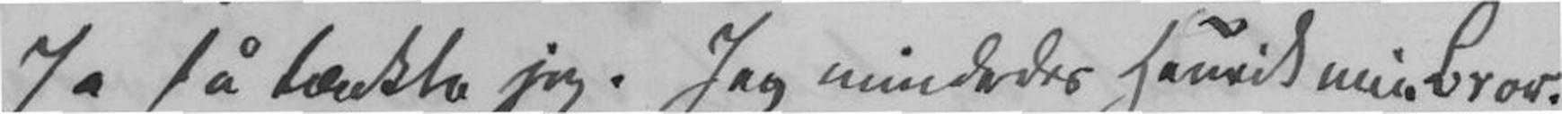Ja så tænkte jeg. Jeg mindedes Henrik min bror.
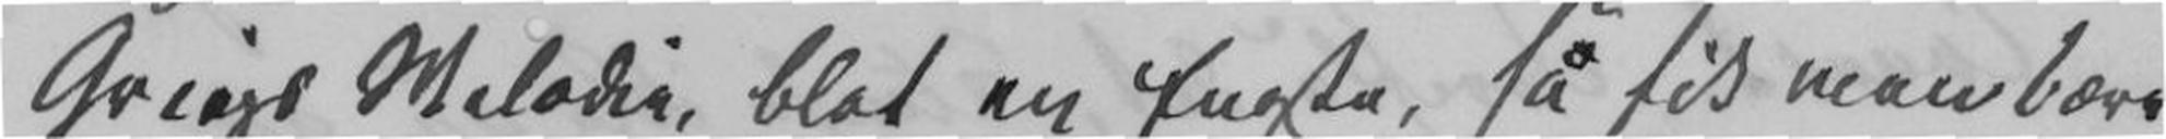Griegs Melodi, blot en Engte, så fik man lære
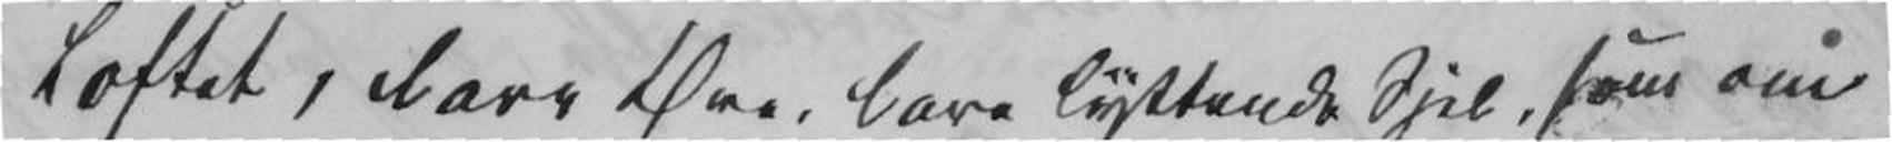Loftet, lave Hus lave lyttende Sjel, som om
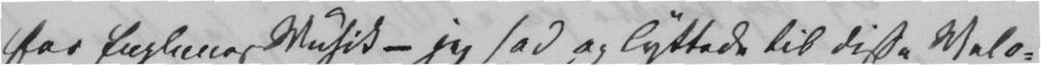for Englenner Musik - Jeg sad og lyttede til disse Melo-
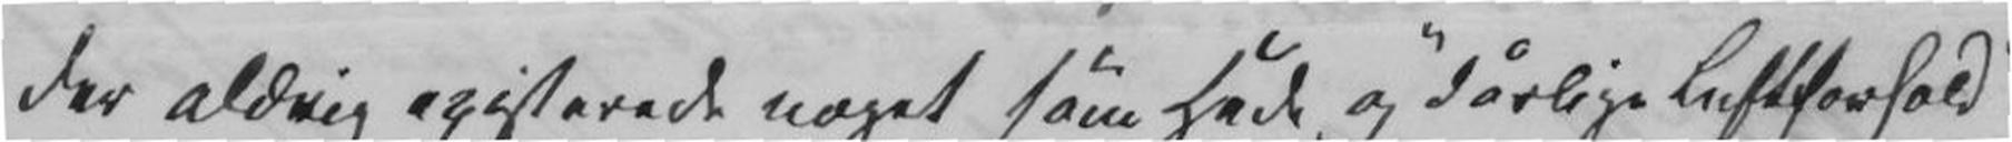der aldrig existerede noget som hede og "dårlige Luftforhold"
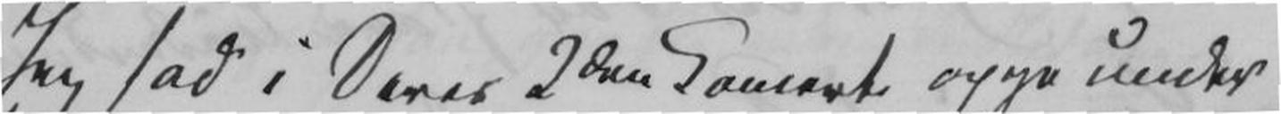Jeg sad i Deres 2den Konsert oppe under
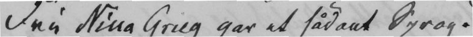Fru Nina Grieg gav et sådant Sprog.
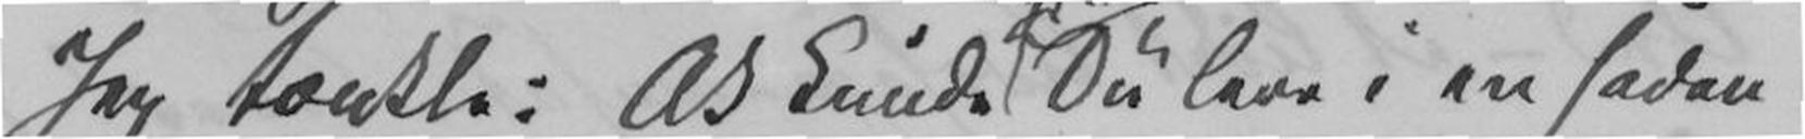Jeg tænkte: Ak kunde Du leve i en sådan
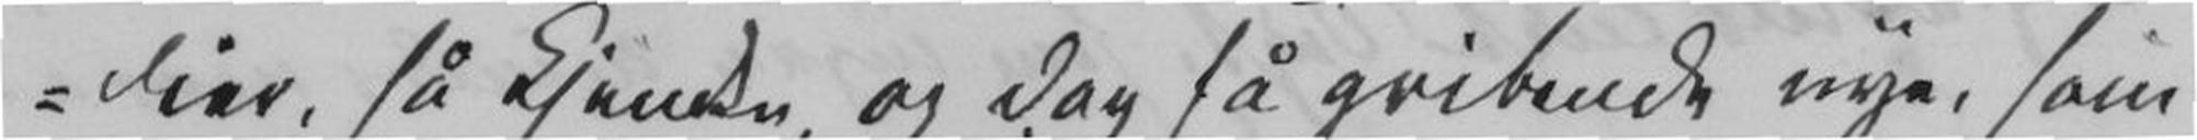dier, så kjendte og dog så gribende nye, som
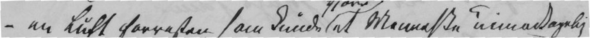- en Luft forresten som Kunde et Menneske uimodtagelig
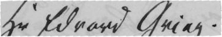Hr Edvard Grieg!
